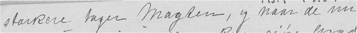stærkere tager Magten, og naar de nu
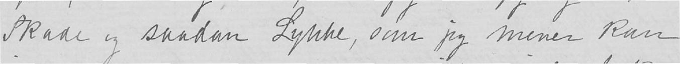Skade og saadan Lykke, som jeg mener kan
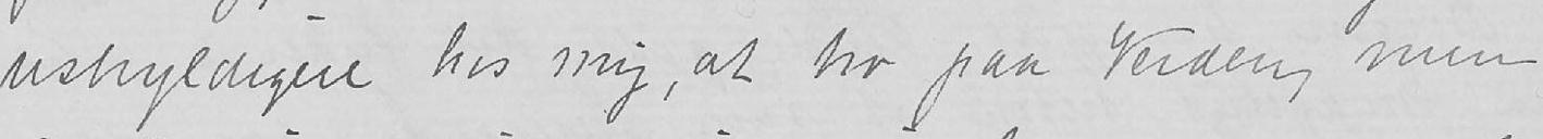uskyldigere hos mig, at tro paa Verden, men
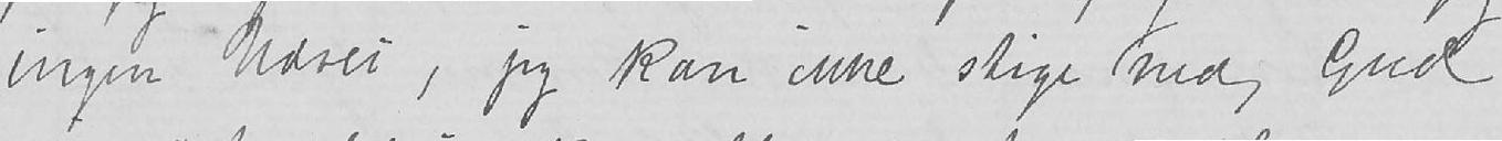ingen Udvei, jeg kan ikke stige ned, Gud
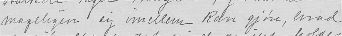mageligen sig imellem kan gjøre, hvad
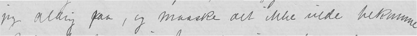jeg aldrig faa, og maaske det ikke vilde bekomme
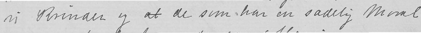vi Kvinder og at de som har en sædelig Moral
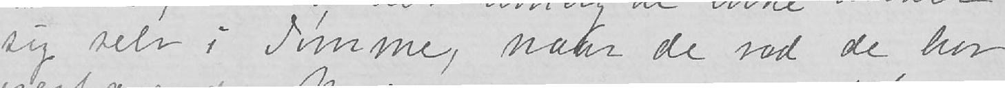sig selv i Tømme, naar de ved de har
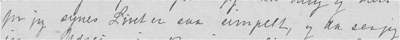for jeg synes Livet er saa simpelt, og da ser jeg
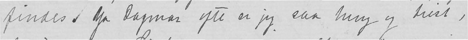findes. Ja Dagmar ofte er jeg saa tung og trist,
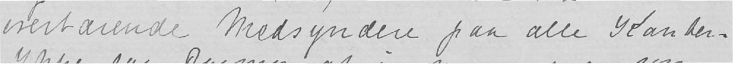taende Medsyndere paa alle Kanter.
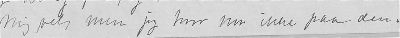mig vel, men jeg tror nu ikke på den.
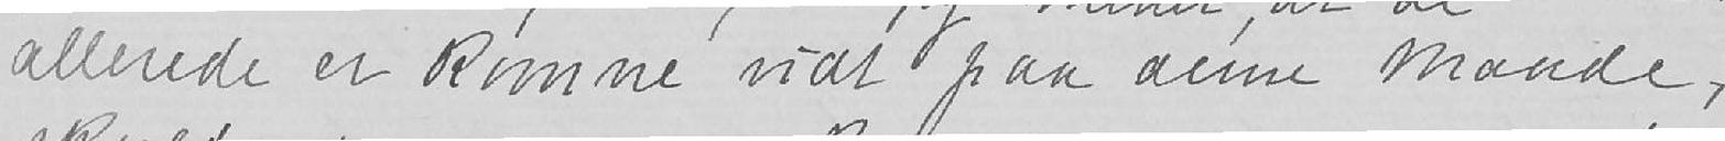allerede er komne vidt paa denne Maade,
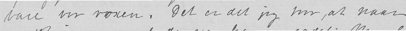bare var voxen. Det er det jeg tror, at naar
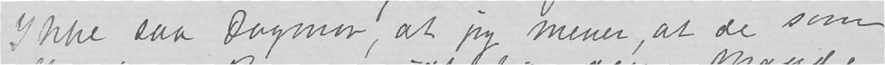Ikke saa Dagmar, at jeg mener, at de som
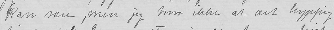kan vare, men jeg tror ikke at det hyppig
